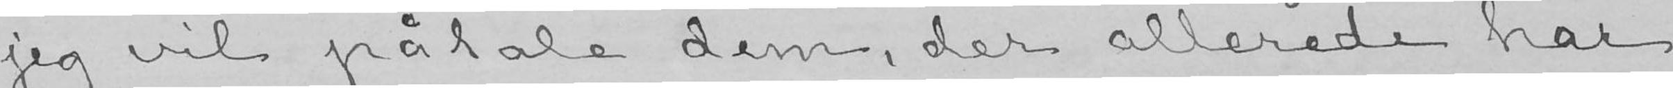jeg vil påtale dem, der allerede har
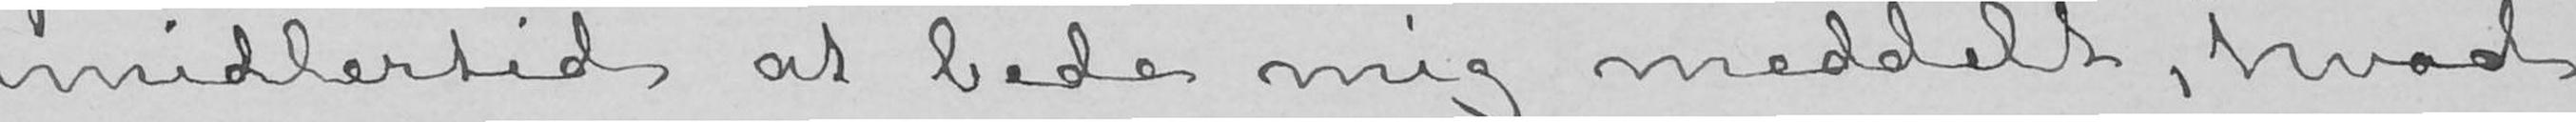imidlertid at bede mig meddelt, hvad
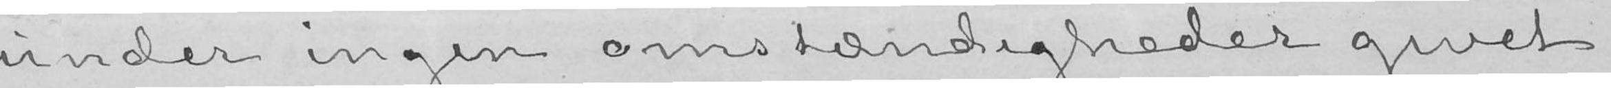under ingen omstændigheder givet
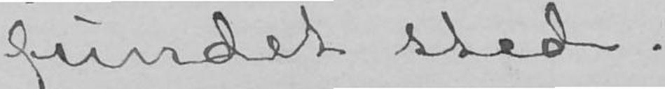fundet sted.
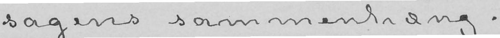sagens sammenhæng.
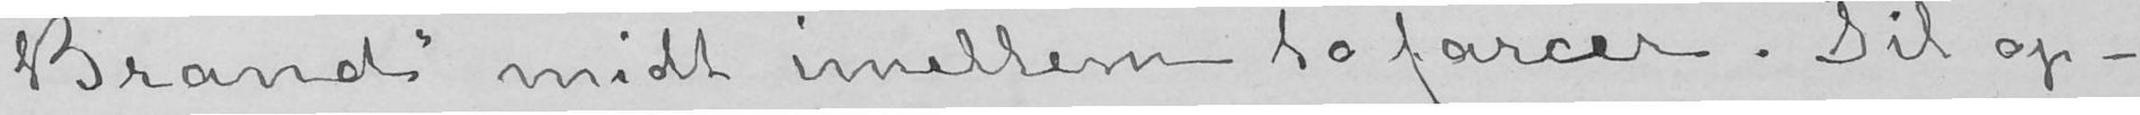«Brand» midt imellem to farcer. Til op-
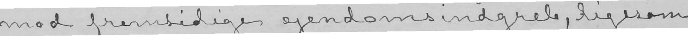mod fremtidige ejendomsindgreb, ligesom
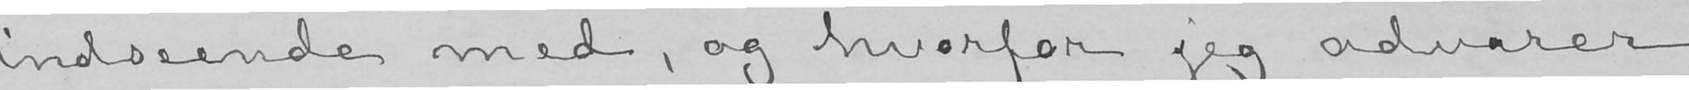indseende med, og hvorfor jeg advarer
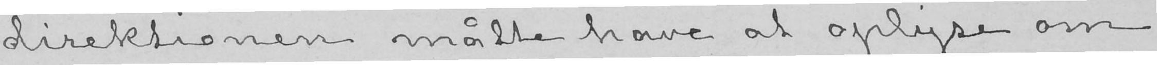direktionen måtte have at oplyse om
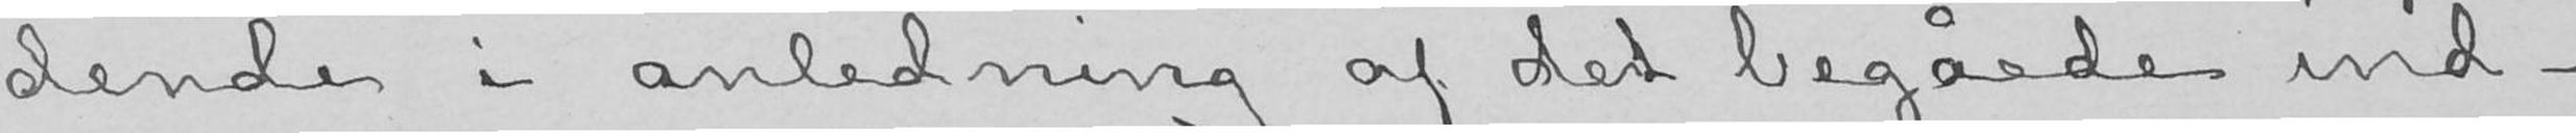dende i anledning af det begåede ind-
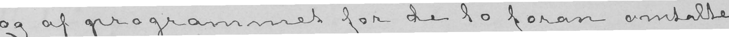og af programmet for de to foran omtalte
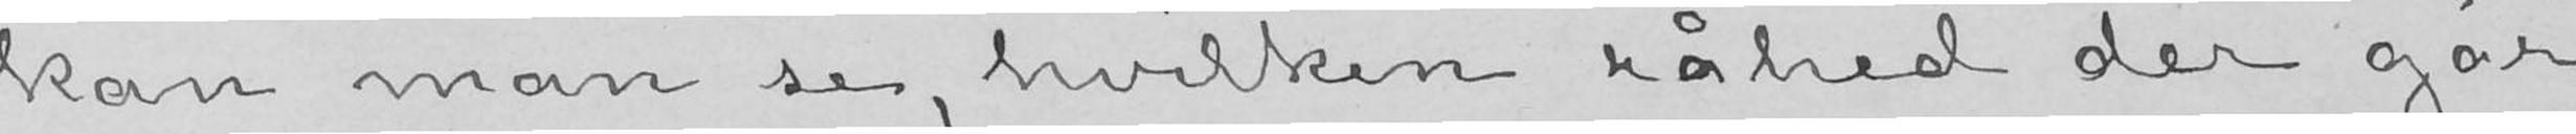kan man se, hvilken råhed der gør
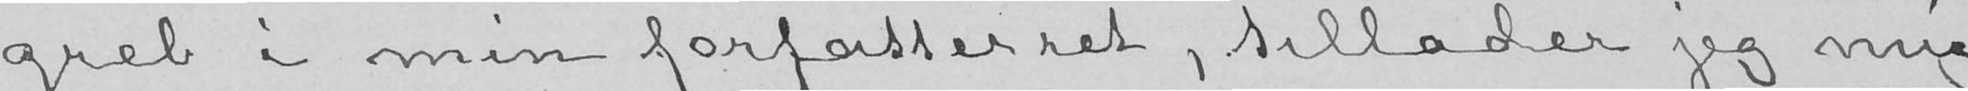greb i min forfatterret, tillader jeg mig
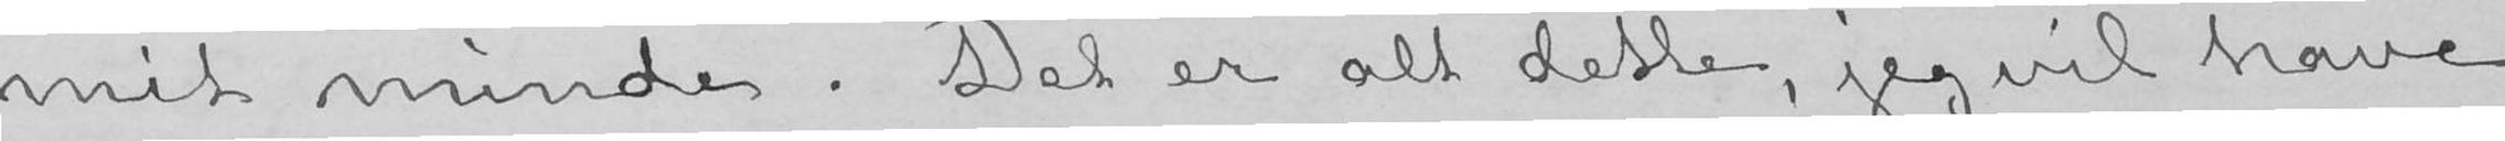mit minde. Det er alt dette, jeg vil have
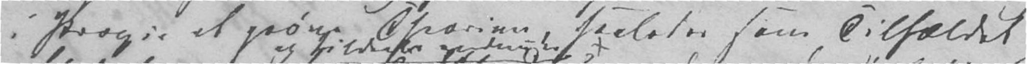i Praxis at prøve Theorien, saaledes som Tilfældet
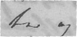ter og
